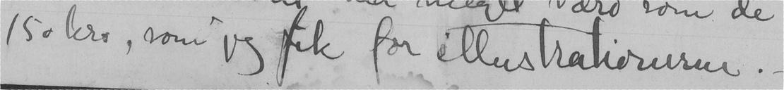150 kro, som jeg fik for illustrationerne. -
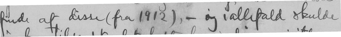finde af disse (fra 1912), - og iallefald skulde
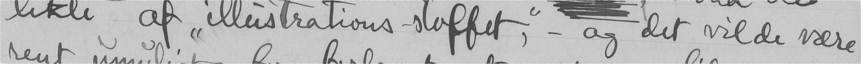likte af "illustrations stoffet"; - og det vilde være
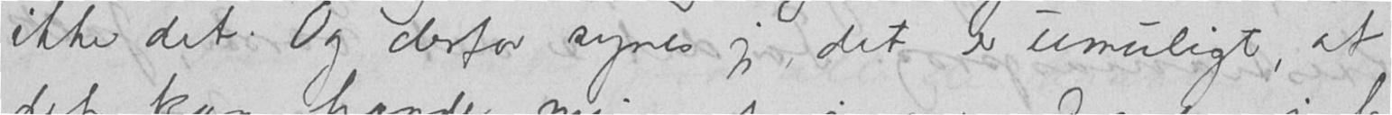ikke det. Og derfor synes jeg, det er umuligt, at
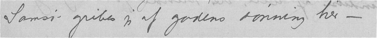Samsø gribes jeg af gadens dønning her -
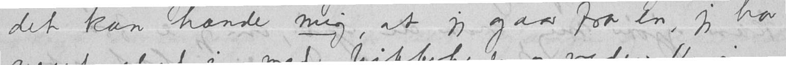det kan hænde mig at jeg gaar fra en jeg har
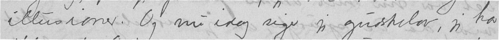illusioner. Og nu idag siger jeg gudskelov, jeg har
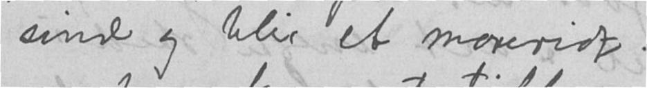sind og blir et mareridt.
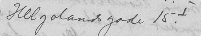Helgalandsgade 15.I
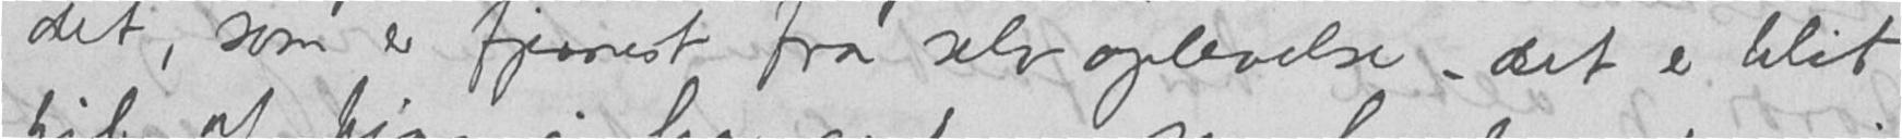det, som er fjernest fra selvoplevelse – det er blit
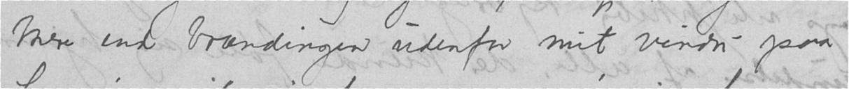Mere end brændingen udenfor mit vindu paa
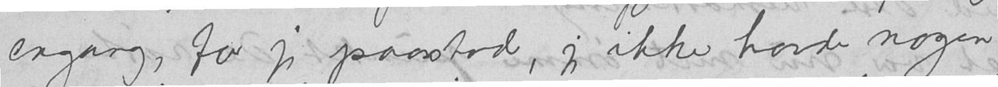engang, for jeg paastod, jeg ikke havde nogen
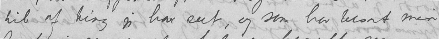til af ting jeg har seet, og som har besat min
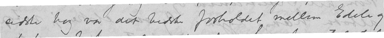sidste bog var det bedste forholdet mellem Edele og
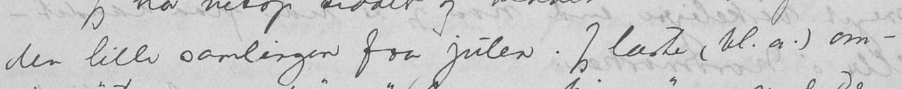den lille samlingen fra julen. Jeg læste (bl.a.) om-
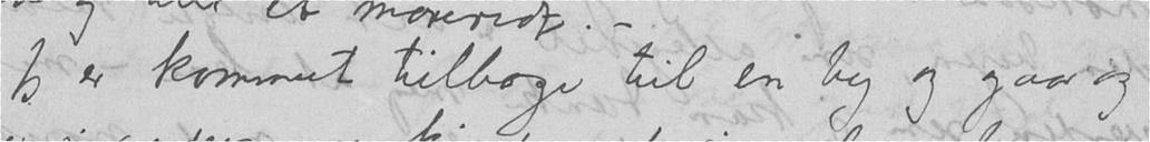Jeg er kommet tilbage til en by og gaar og
